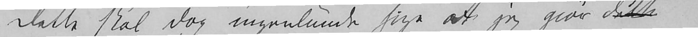Dette skal dog ingenlunde sige at jeg gjør dette
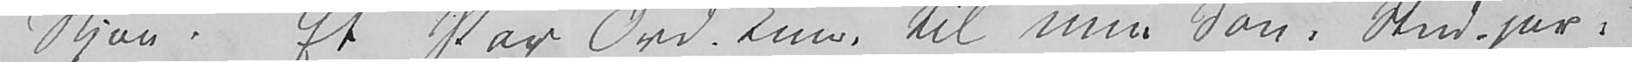Skjøn. Et Par Ord, kun, til min Søn, Stud.jur.
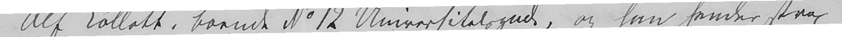Alf Collett, boende No 12 Universitetsgade, og han sender strax
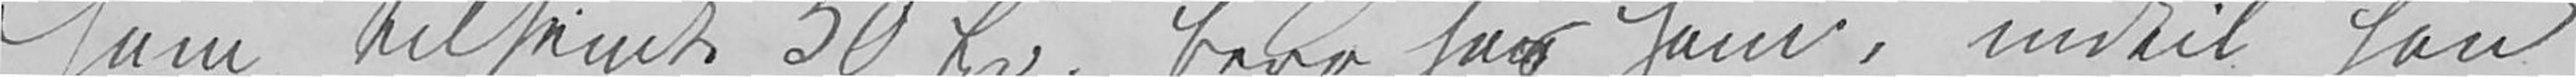ham tilsendte 30 Spd. bero hos ham, indtil han
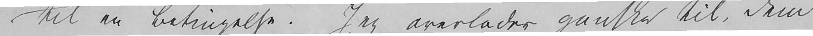til en Betingelse. Jeg overlader ganske til, Dem
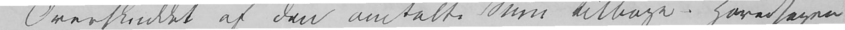Overskuddet af den omtalte Sum tilbage. Hovedsagen
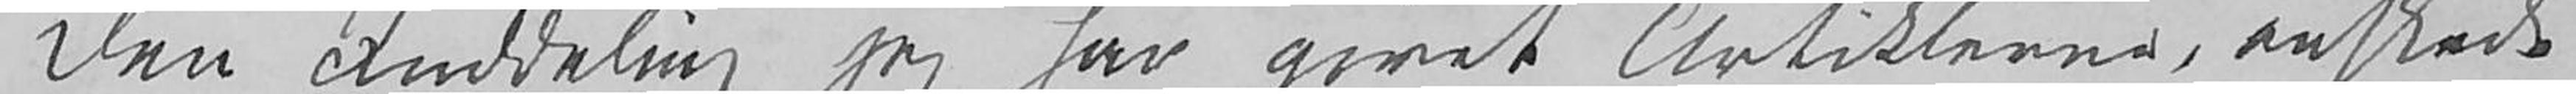Den Inddeling jeg har givet Artiklerne, ønskede
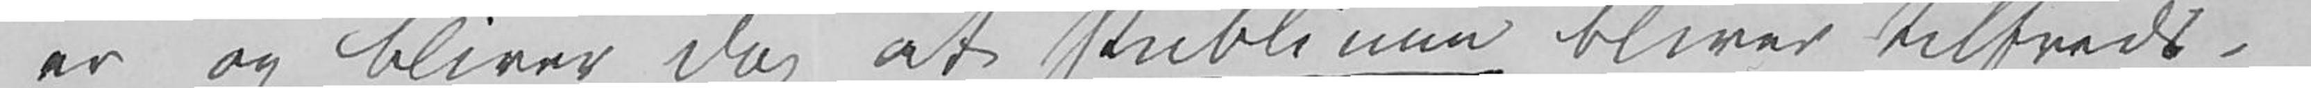er og bliver dog at Publicum bliver tilfreds.
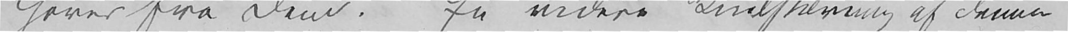hører fra Dem. En videre Indstævning af denne
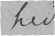hed
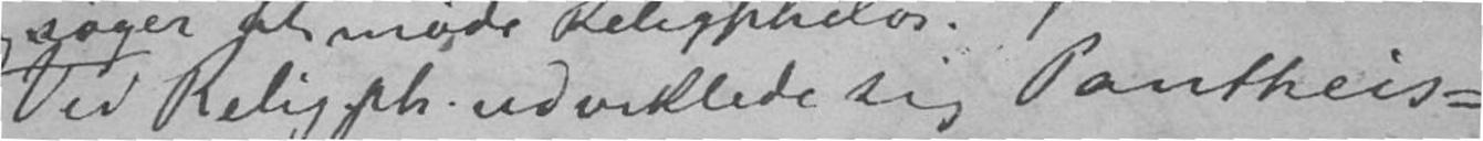Ved Religph. udviklede sig Pantheis-
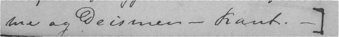me og Deismen – Kant. –]
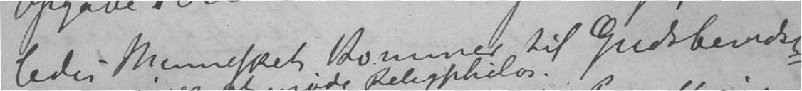ledes Mennesket kommer til Gudsbevidst
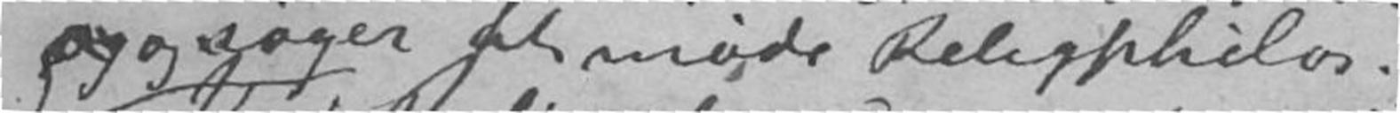og søger at møde Religphilos.
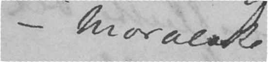– Moralske –
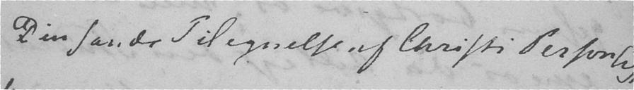Den sande Tilegnelse af Christi Personlighed
